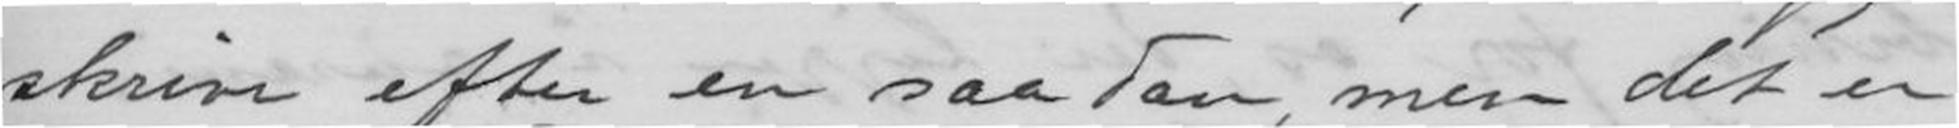skrive efter en saadan, men det er
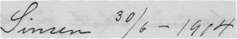Sinsen 30/6-1904
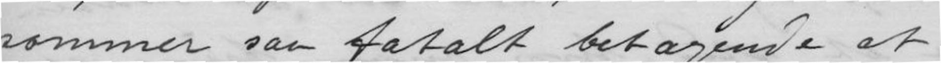sommer saa fatalt betagende at
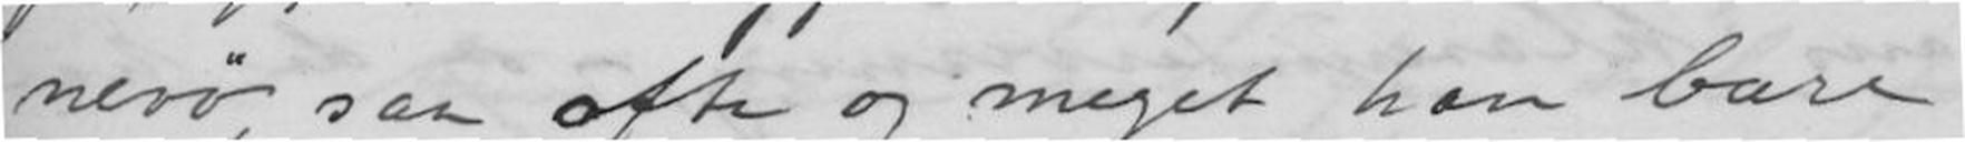nevø, saa ofte og meget han bare
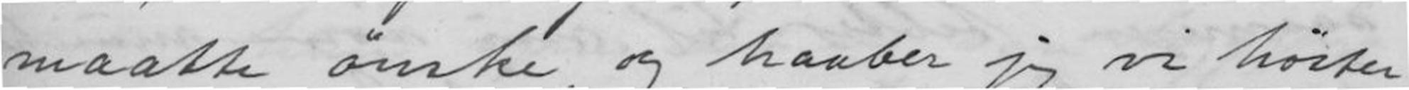maatte ønske, og haaber jeg vi høster
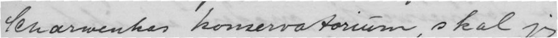Scharwenkas konservatorium, skal jeg
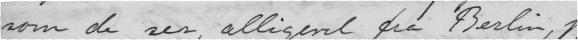som de ser, alligevel fra Berlin, jeg
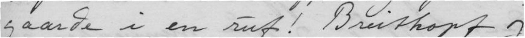gaarde i en ruf! Breitkopf og
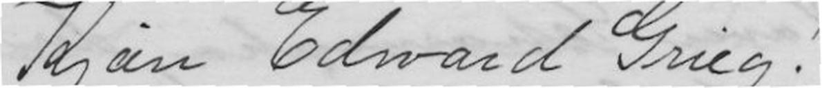Kjare Edvard Grieg!
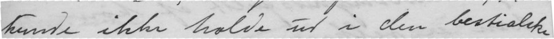kunde ikke holde ud i den bestialske
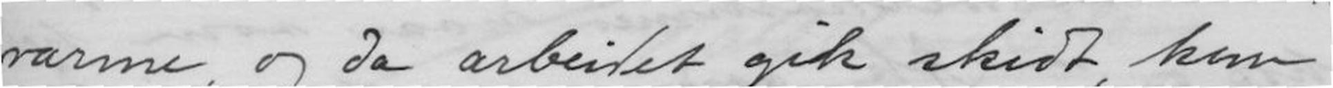varme, og da arbeidet gik skidt, kom
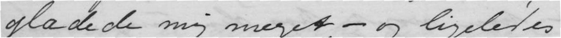glædede mig meget, - og ligeledes
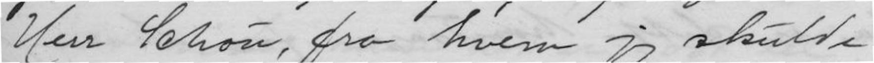Herr Schou, fra hvem jeg skulde
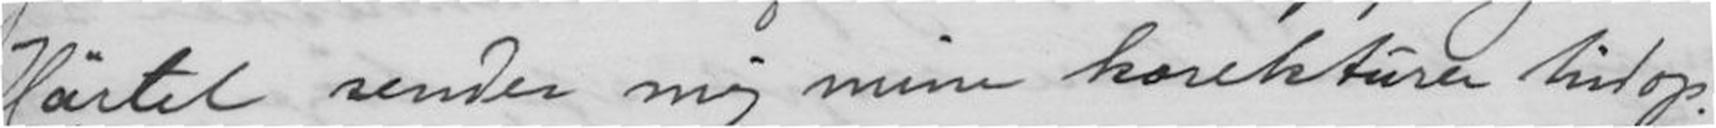Härtel sender mig mine korekturer hidop.
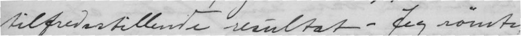tilfredsstillende resultet. - Jeg rømte
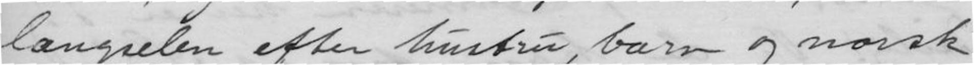længselen efter hustru, barn og norsk
